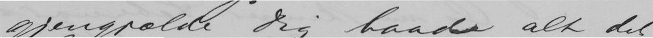gjengjælde Dig baade alt det
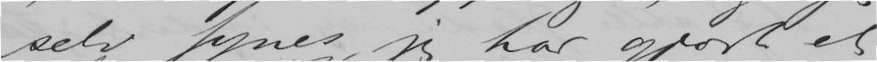selv synes, jeg har gjort et
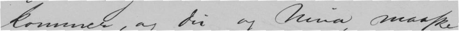kommer, og Du og Nina maaske
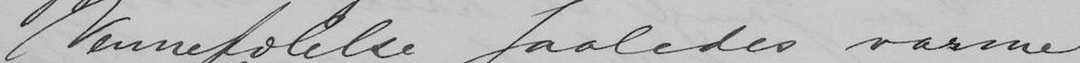Vennefølelse saaledes varme
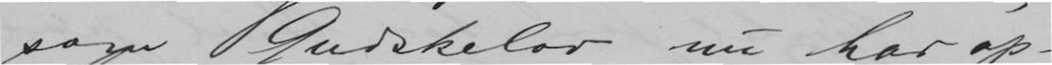som Gudskelov nu har op-
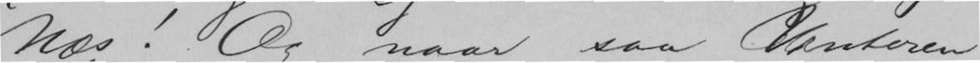Næs! Og naar saa Vinteren
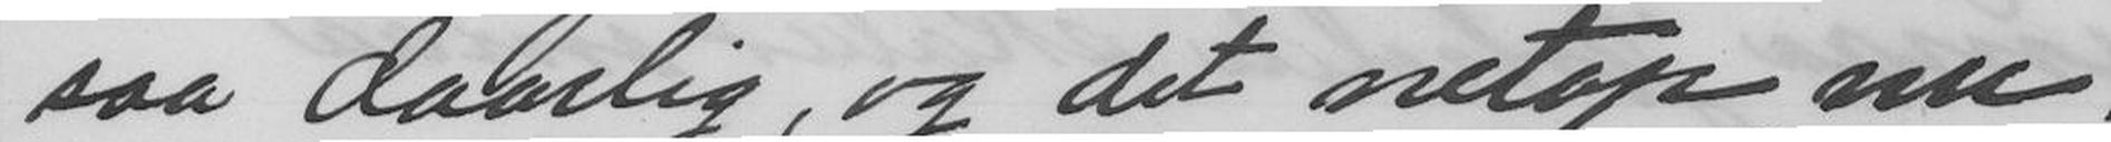saa daarlig, og det netop nu
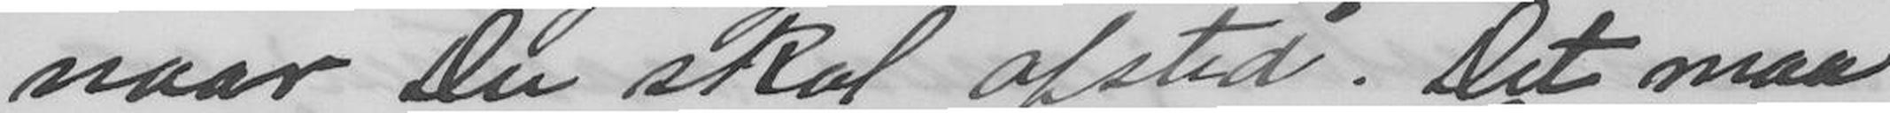naar Du skal afsted. Det maa
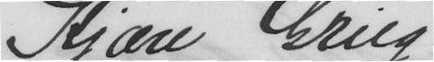Kjære Grieg!
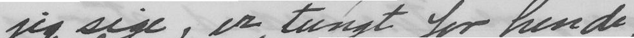jeg sige, er tungt for hende
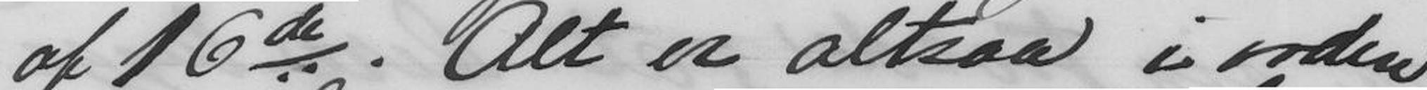af 16de. Alt er altsaa i orden.
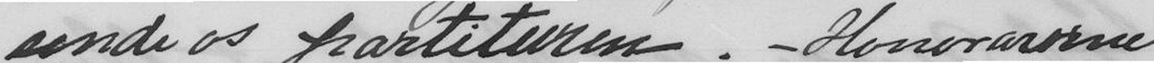sende os partituren. - Honorarerne
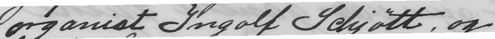organist Ingolf Schjøtt, og
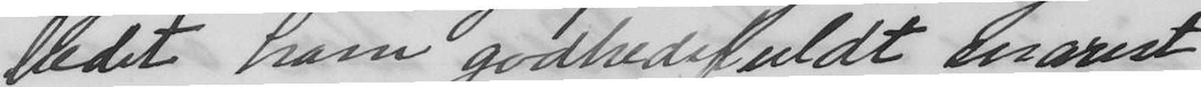bedet ham godhedsfuldt snarest
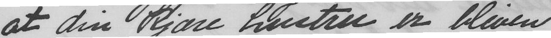at din kjære hustru er bliven
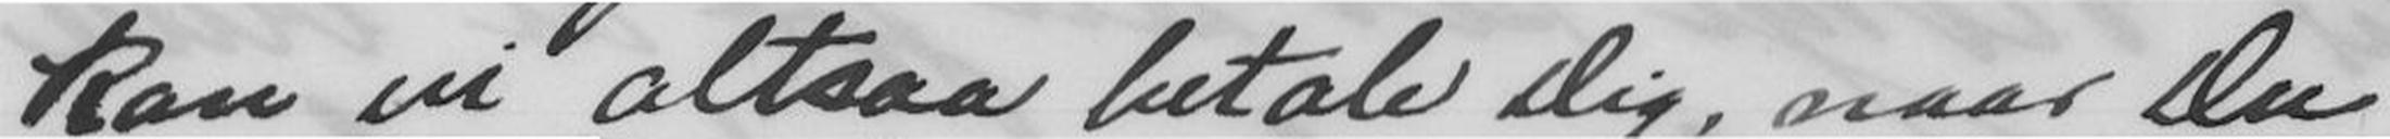kan vi altsaa betale Dig, naar Du
